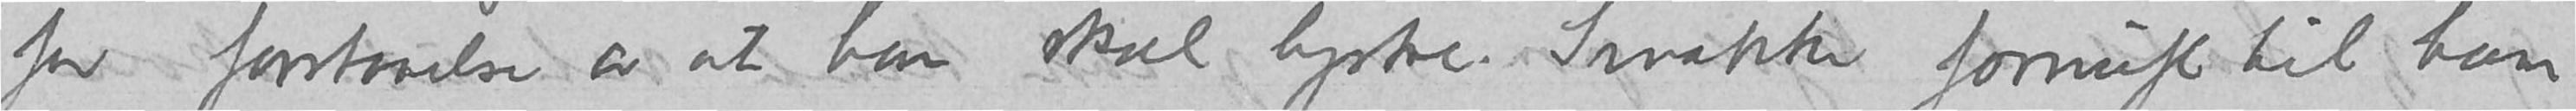for forstaaelse av at han skal lystre. Snakke fornuft til ham
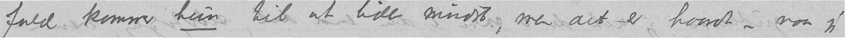fald kommer hun til at lide mindst, men det er haardt – naar jeg
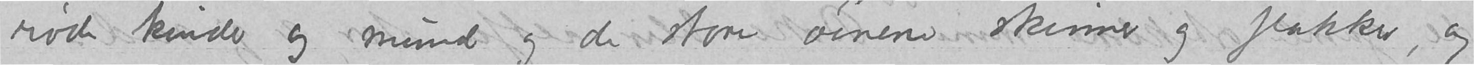røde kinder og mund og de store øinene skinner og flakker, og
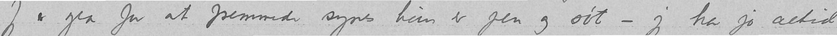Jeg er gla for at fremmede synes hun er pen og søt – jeg har jo altid
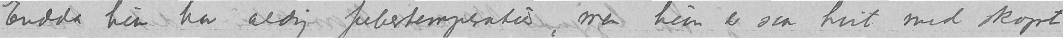Endda hun har aldrig febertemperatur, men hun er saa hvit med skarpt
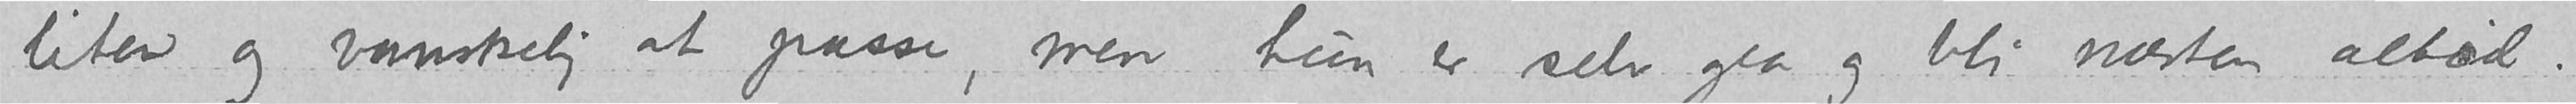liten og vanskelig at passe, men hun er selv gla og bli næsten altid.
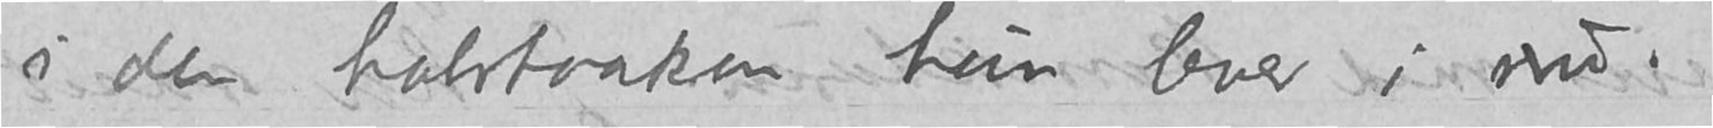i den halvtaaken hun lever i nu.
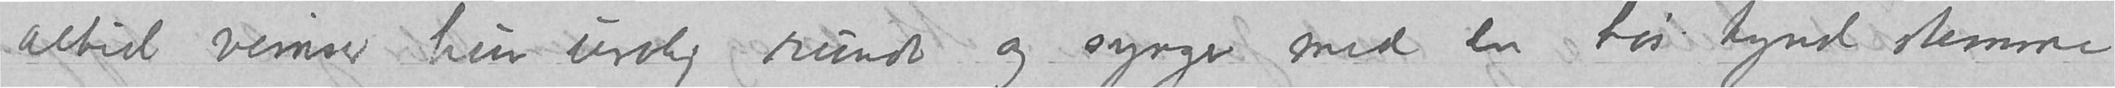altid vimser hun urolig rundt og synger med en hes tynd stemme
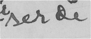ser de
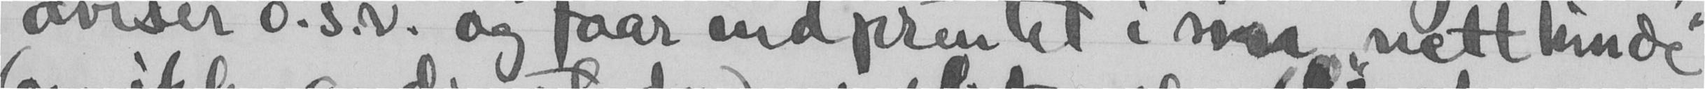aviser o.s.v. og faar indprentet i sin "netthinde"
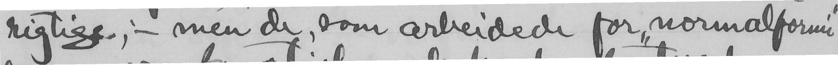rigtige, - men de, som arbeidede for "normalformi"
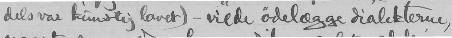dels var kunstig lavet) - vilde ødelægge dialikterne,
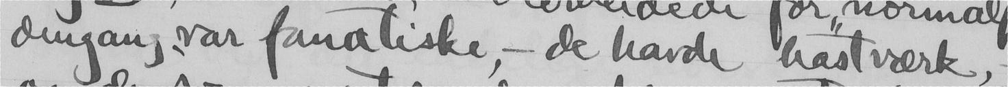dengang var fanatiske - de havde hastværk, -
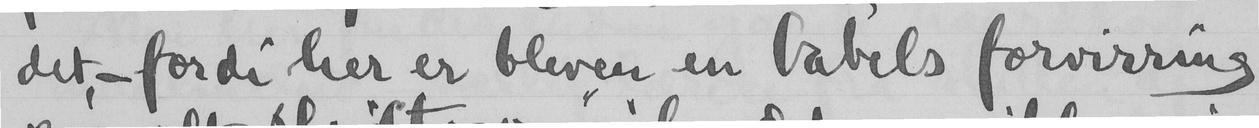det,- fordi her er bleven en babels forvirring
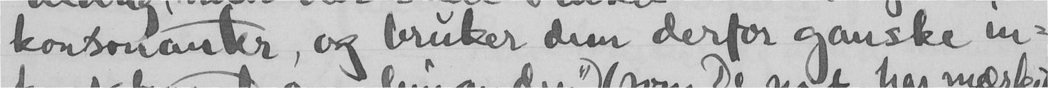konsonanter, og bruker dem derfor ganske in-
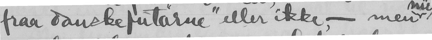fraa danskefutarne eller ikke - men
